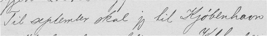Til september skal jeg til Kjøbenhavn
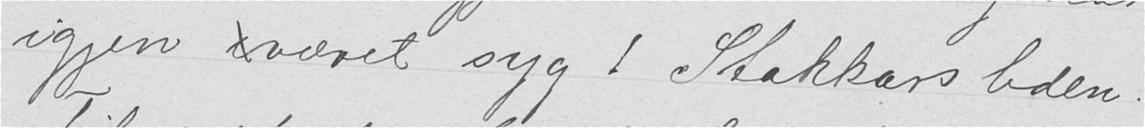igjen Vværet syg! Stakkars liden.
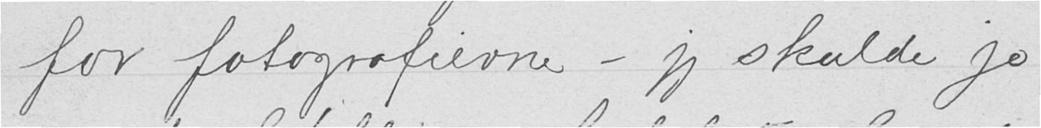for fotografierne – jeg skulde jo
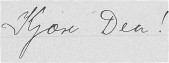Kjære Dea!
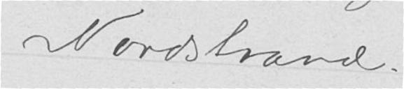Nordstrand.
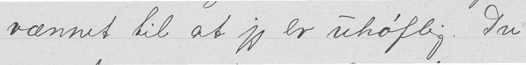vænnet til at jeg er uhøflig. Du
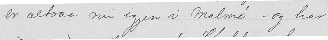er altsaa nu igjen i Malmø - og har
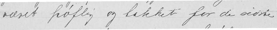været høflig og takket for de sidste
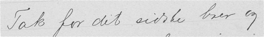Tak for dit sidste brev og
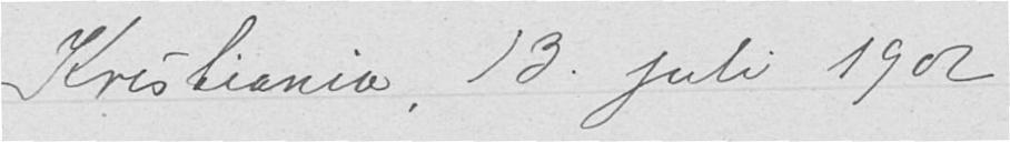Kristiania, 13. juli 1902
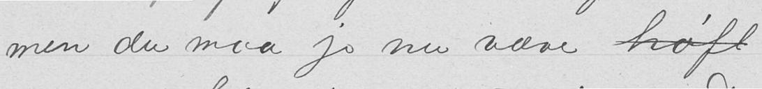men du maa jo nu være høfl,
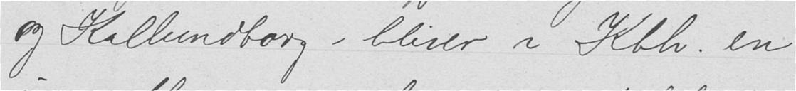og Kallundborg – bliver i Kbh. en
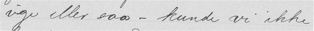uge eller saa – kunde vi ikke
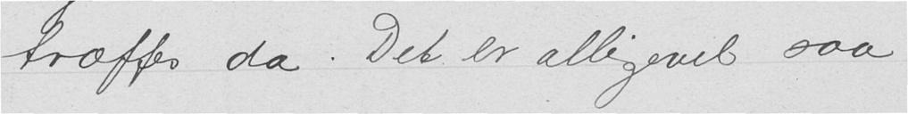træffes da. Det er alligevel saa
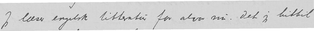Jeg læser engelsk litteratur for alvor nu. Det jeg hittil
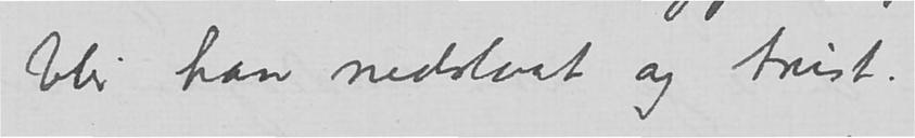blir han nedslaat og trist.
